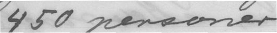450 personer
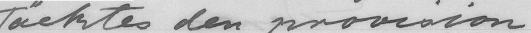täcktes den provision
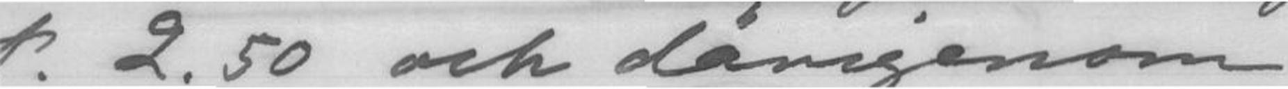til 2.50 och därigenom
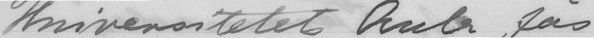Universitetets Aula fås
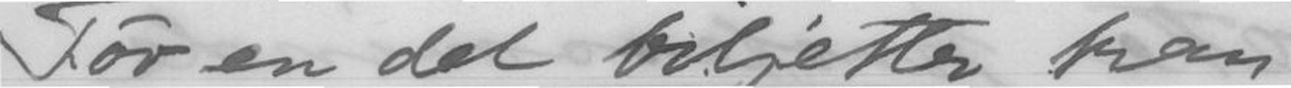För en del biljeter kan
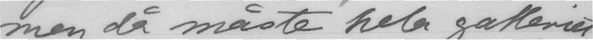men då måste hela galleriet
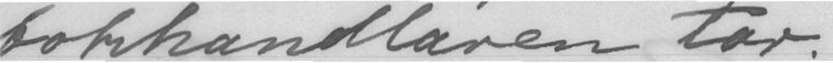bokhandlaren tar.
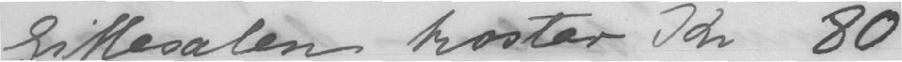Spillesalen koster kr. 80
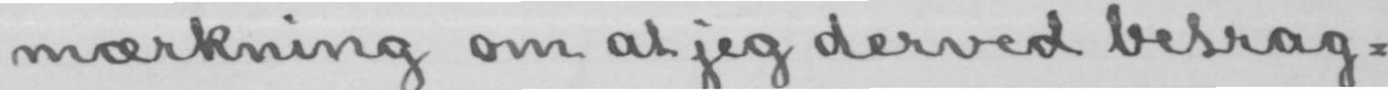mærkning om at jeg derved betrag-
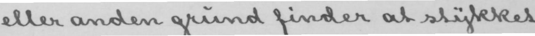eller anden grund finder at stykket
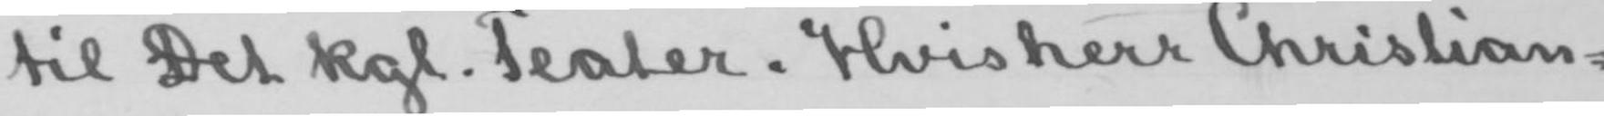til Det kgl. Teater. Hvis herr Christian-
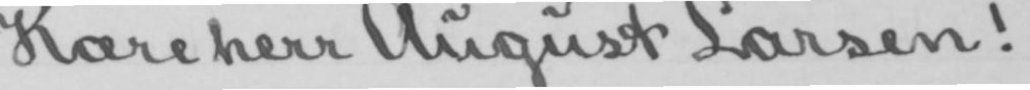Kære herr August Larsen!
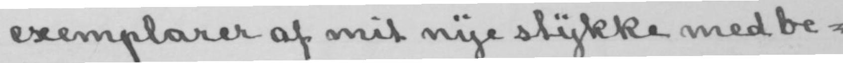exemplarer af mit nye stykke med be-
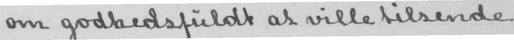om godhedsfuldt at ville tilsende
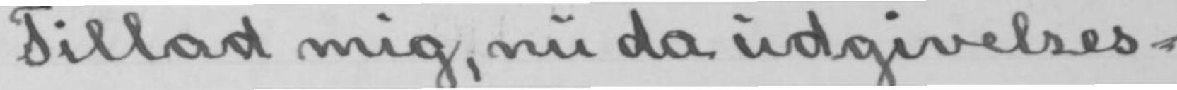Tillad mig, nu da udgivelses-
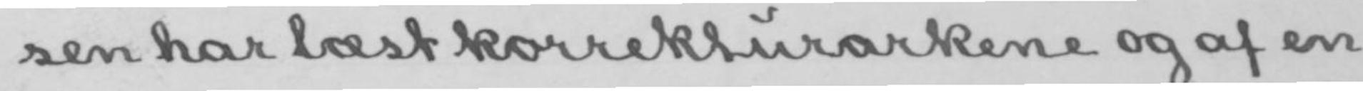sen har læst korrekturarkene og af en
